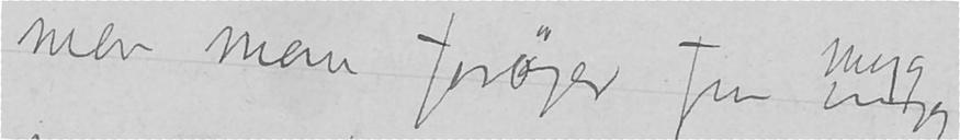men man forøger fra myg
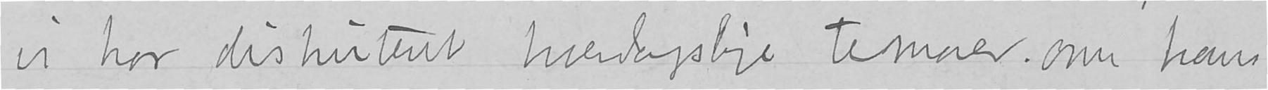vi har diskuteret hverdagslige temaer, om hans
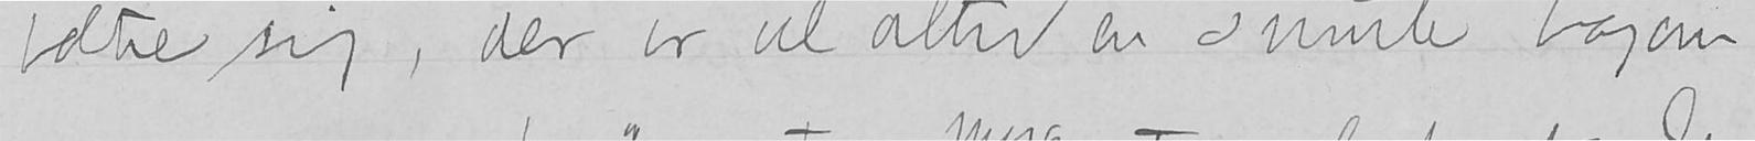boltre sig, der er vel altid en smule bagom,
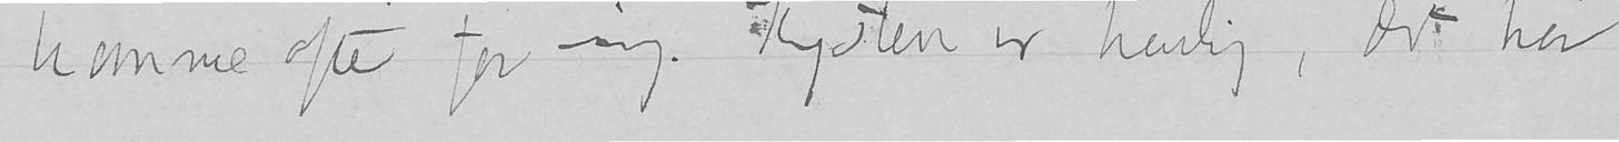komme ofte for mig. Kysten er herlig, det har
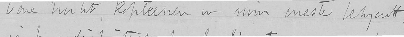bare hvilet, Kapteinen er min eneste bekjendt,
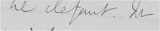til elefant. Det
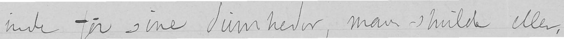inde for sine dumheder, man skulde eller,
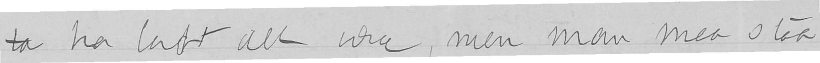ta ha latt det være, men man maa staa
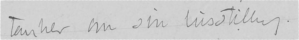tanker om sin livsstilling.
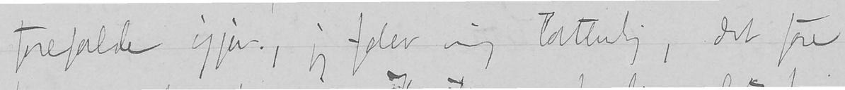forefalde igjen, jeg føler mig latterlig, det fore
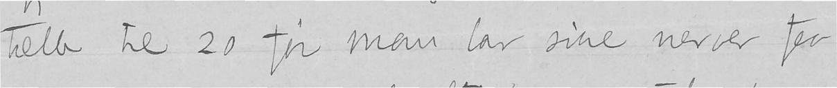tælle til 20 før man lar sine nerver faa
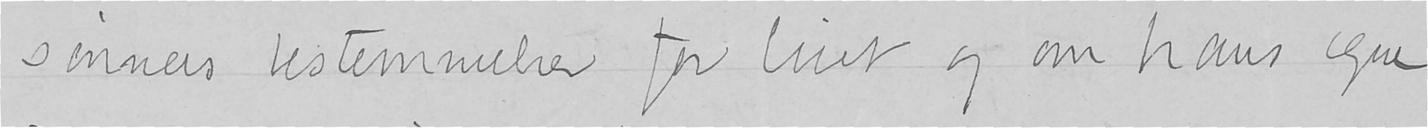sønners bestemmelser for livet og om hans egne
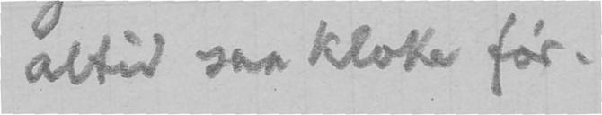altid saa kloke før.
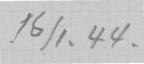16/1. 44.
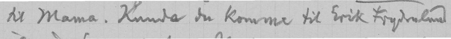til Mama. Kunde du komme til Erik Frydenlund
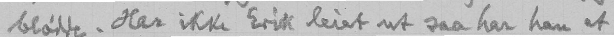blødde. Har ikke Erik leiet ut saa har han et
